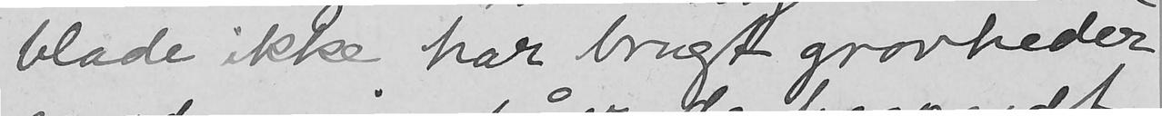blade ikke har brugt grovheder
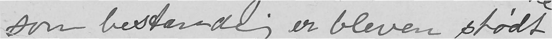som bestandig er bleven stødt
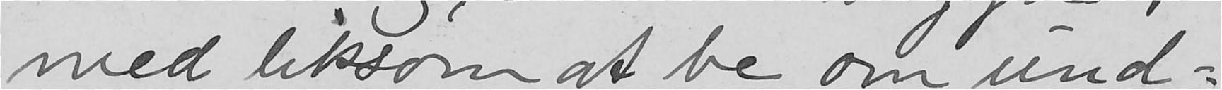med liksom at be om und-
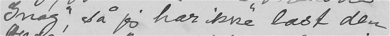Gnag", så jeg har ikke læst den
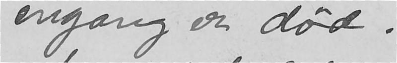engang eg død.
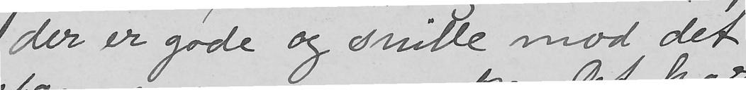der er gode og snille mod det
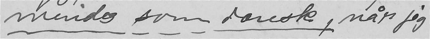mindes som dansk, når jeg
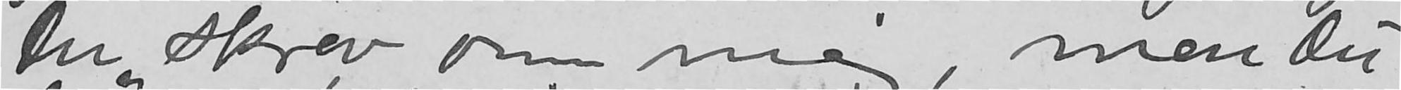Du skrev om mig, men Du
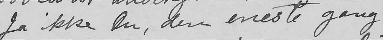Ja ikke Du, den eneste gang
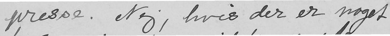presse. Nej, hvis der er noget
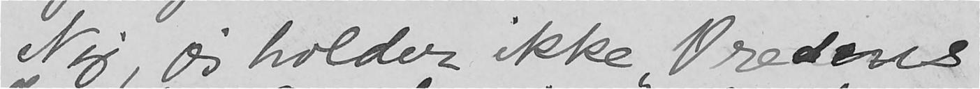Nej, jeg holder ikke "Vredens
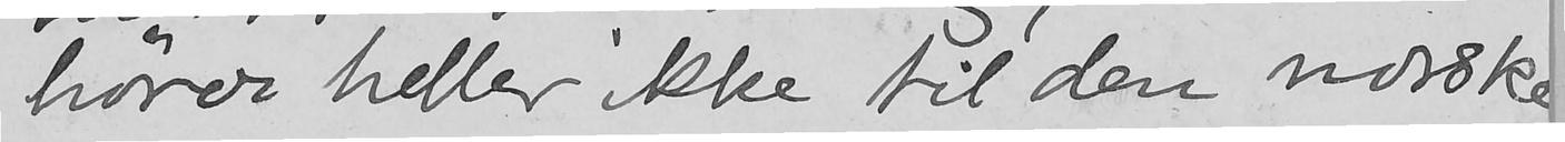hører heller ikke til den norske
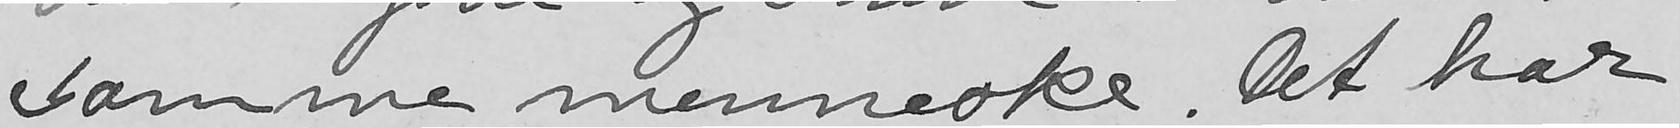samme menneske. Det har
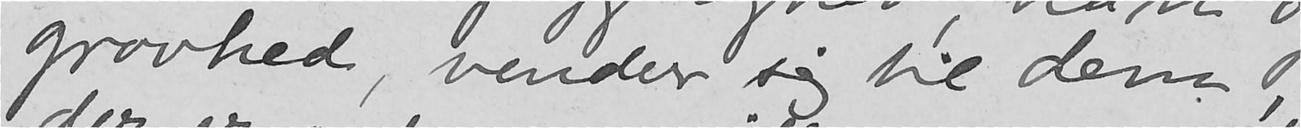grovhed, vender sig til dem,
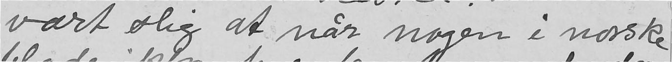vært slig at når nogen i norske,
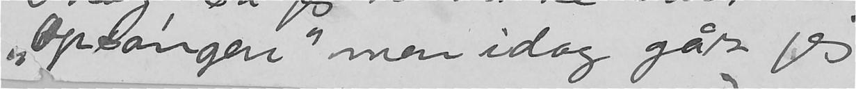"Opsangen" men idag går jeg
